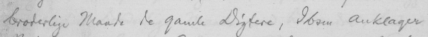broderlige Maade de gamle Digtere, Ibsen anklager
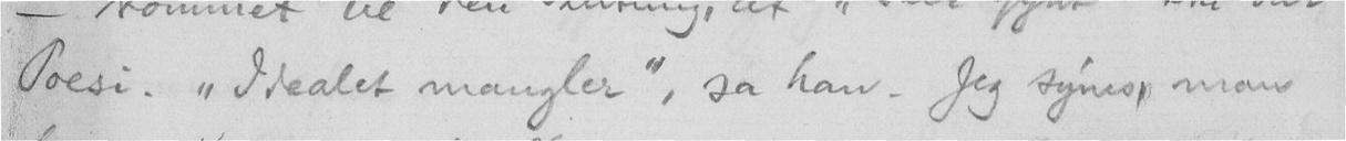Poesi. "Idealet mangler", sa han. Jeg synes, man
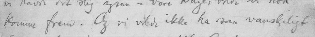komme frem. Og vi vilde ikke ha saa vanskeligt
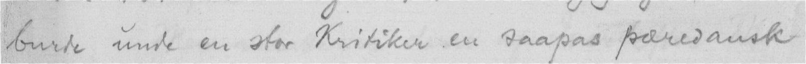kunde unde en stor Kritiker en saapas pæredansk
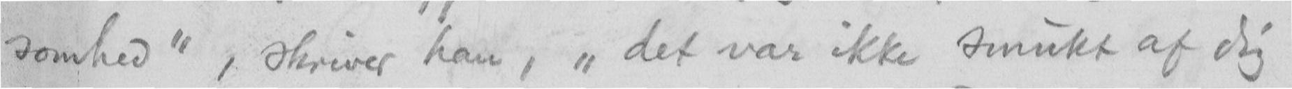somhed", skriver han, "det var ikke smukt af dig
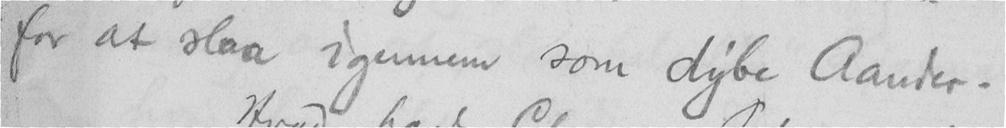for at slaa igennem som dybe Aander.
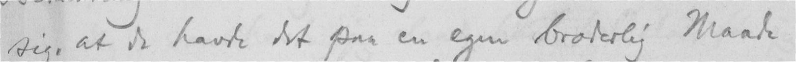sig, at de havde det paa en egen broderlig Maade
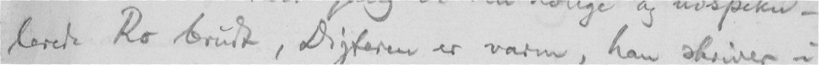lerede Ro brudt, Digteren er varm, han skriver i
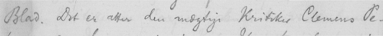Blad. Det er atter den mægtige Kritiker Clemens Pe-
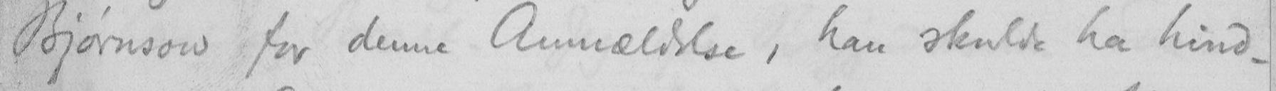Bjørnson for denne Anmældelse, han skulde ha hind-
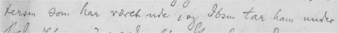tersen som har været ude, og Ibsen tar ham under
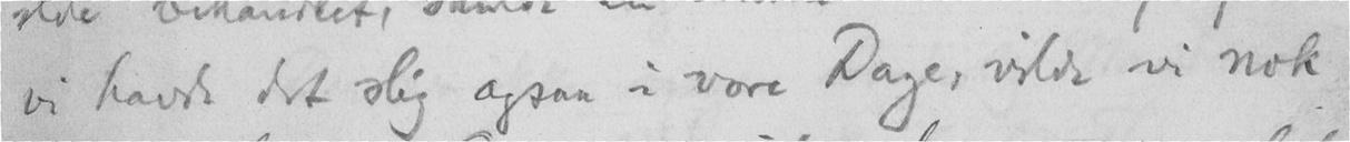vi havde det slig ogsaa i vore Dage, vilde vi nok
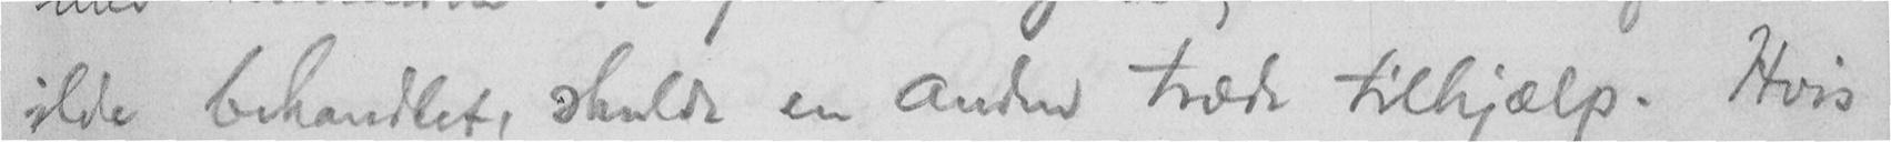ilde behandlet, skulde en anden træde tilhjælp. Hvis
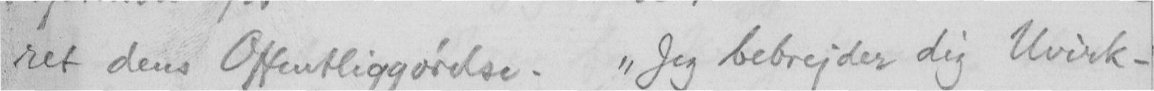ret dens Offentliggørelse. "Jeg bebrejder dig Uvirk-
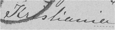Kristiania
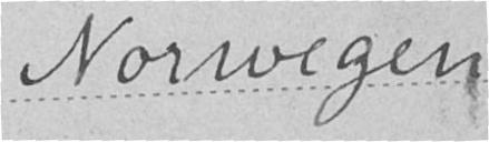Norwegen
